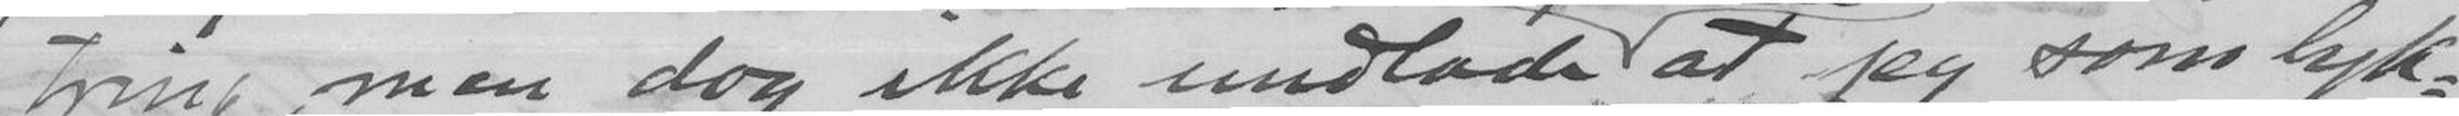tring, men dog ikke undlade at jeg som lyk-
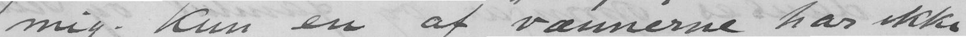mig. Kun en af vænnerne har ikke
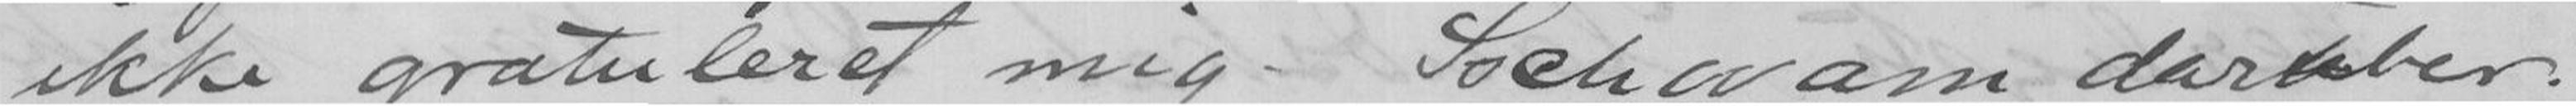ikke gratuleret mig. Schwam darüber!
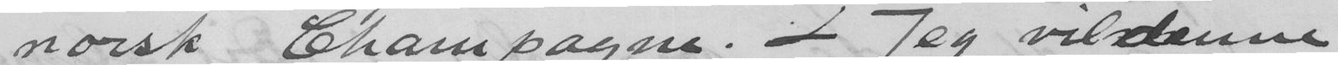norsk Champagne. - Jeg vil denne
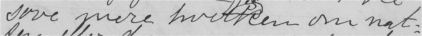sove mere hverken om nat-
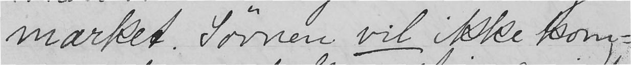mærket. Søvnen vil ikke kom-
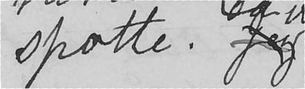spotte. Jeg
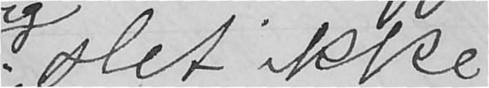slet ikke
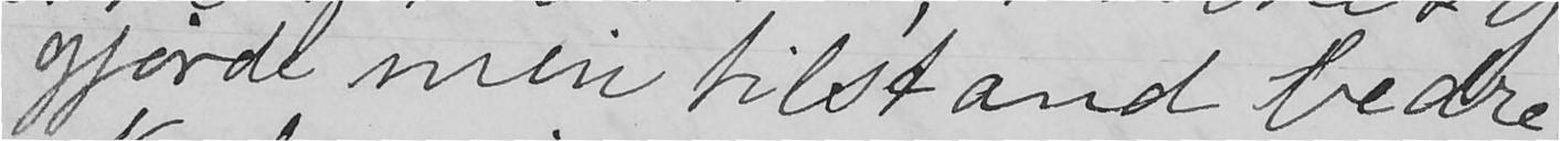gjorde min tilstand bedre.
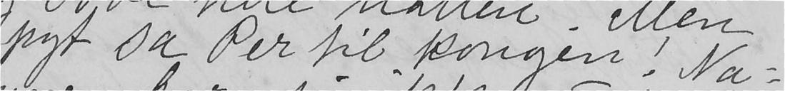pyt sa Per til kongen! Na-
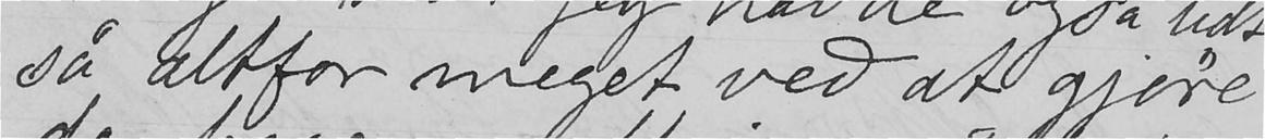så altfor meget ved at gjøre
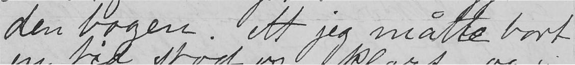den bogen. At jeg måtte bort
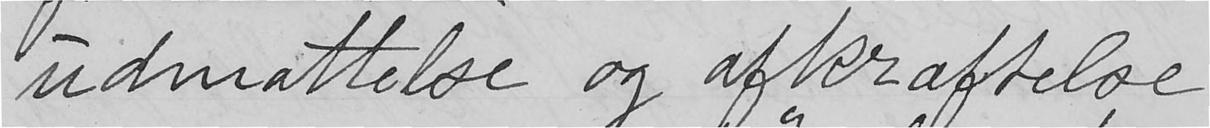udmattelse og afkræftelse
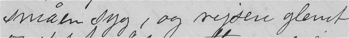småen syg, og rejsen glemt
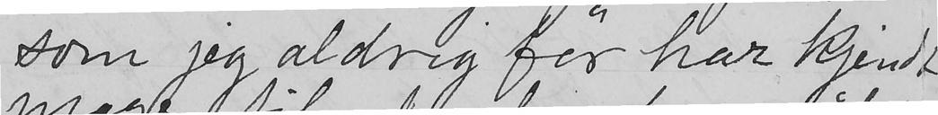som jeg aldrig før har kjendt
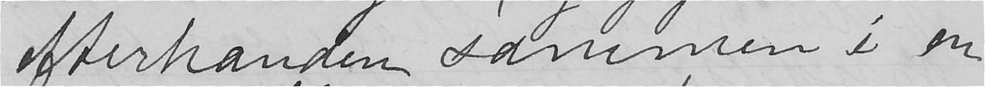efterhanden sammen i en
